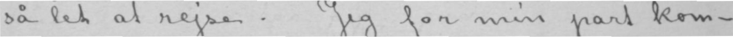så let at rejse. Jeg for min part kom-
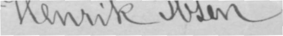Henrik Ibsen.
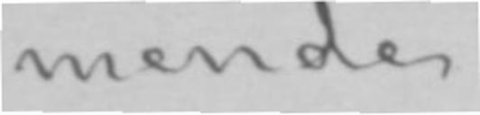mende.
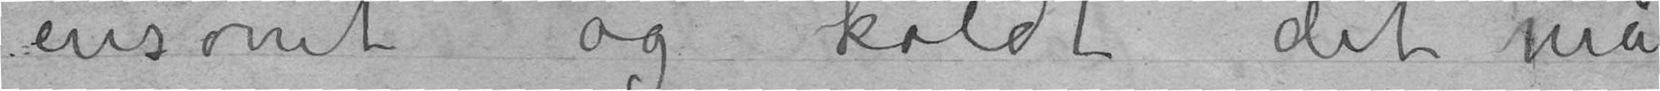ensomt og koldt det må
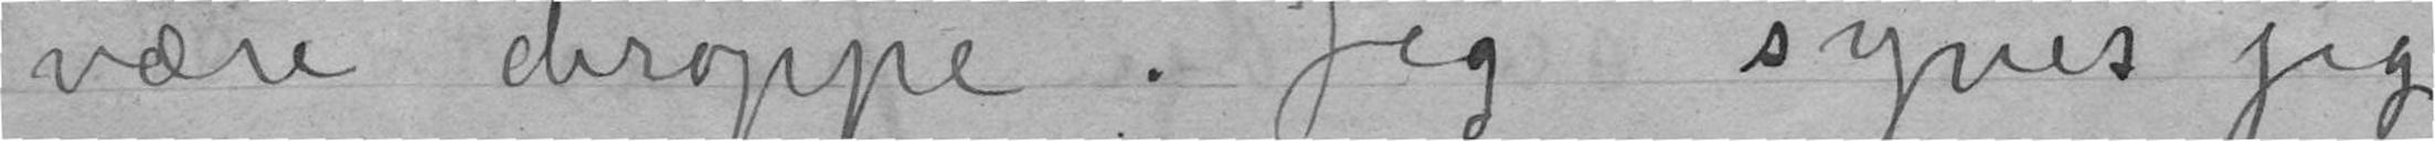være deroppe. Jeg synes jeg
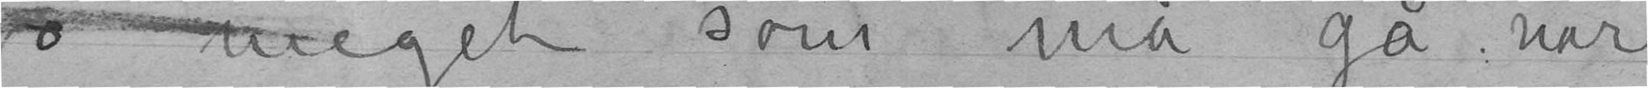jo meget som må gå når
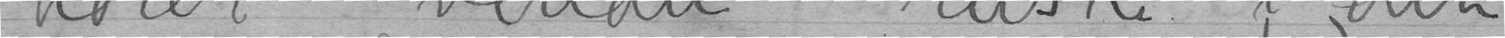hører vinden ruske i den
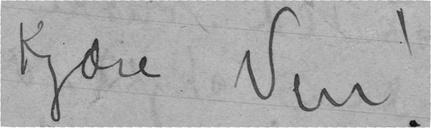Kjære Ven!
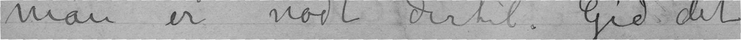man er nødt dertil. Gid det
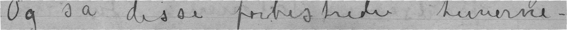Og så disse forbistrede timerne –
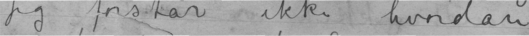Jeg forstår ikke hvordan
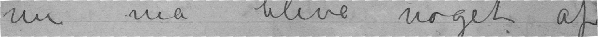nu må blive noget af
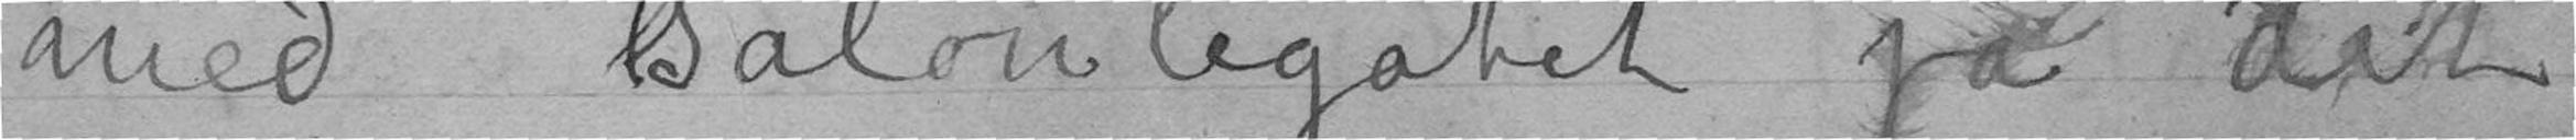med salonlegatet ja det
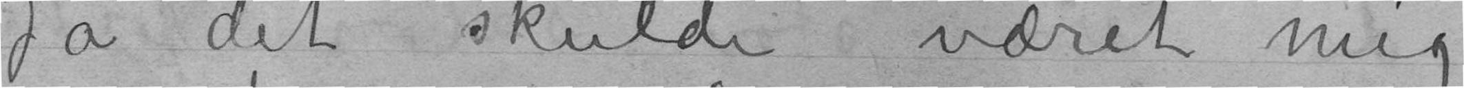Ja det skulde været mig
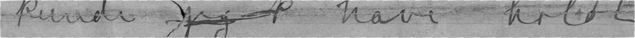jeg kunde jeg k have holdt
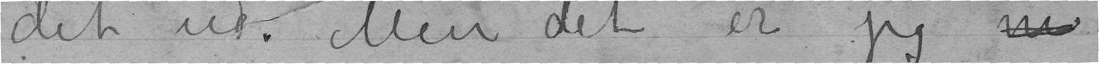det ud. Men det er jeg m
